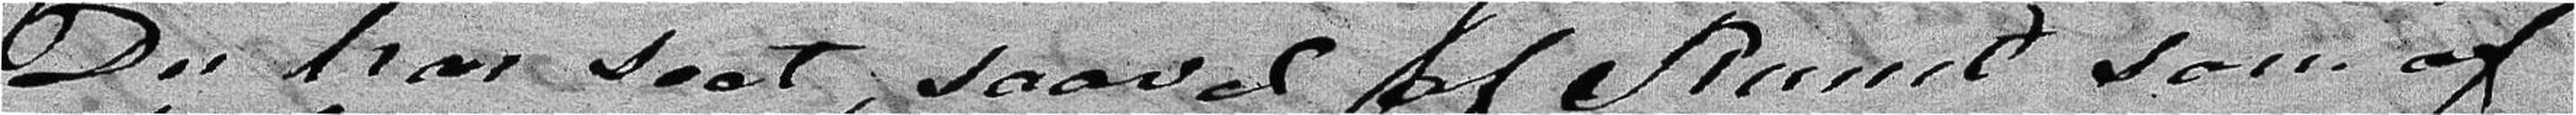Du har seet, saavel af Kunst som af
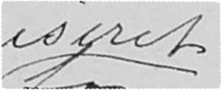iseret
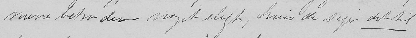mere betro dem noget sligt, hvis de siger det til
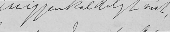uigjenkaldeligt vist,
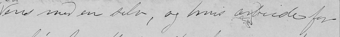ens med en selv, og hvis arbeide for
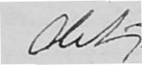det,
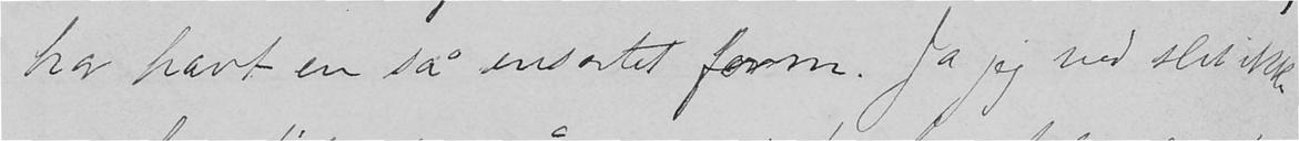har havt en så ensartet form. Ja jeg ved slet ikke
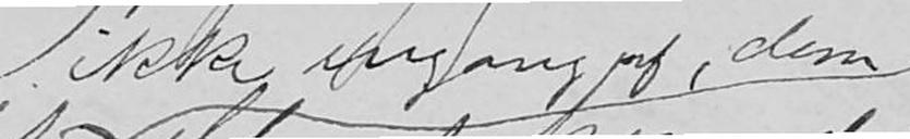ikke ingang af dem
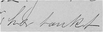har tænkt
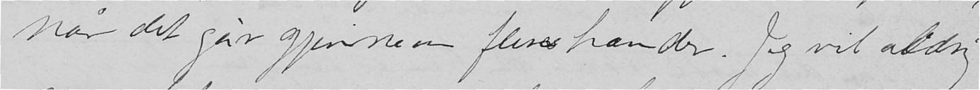når det går gjennem flere hænder. Jeg vil aldrig
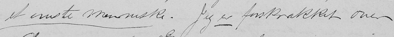et eneste menneske. Jeg er forskrækket over
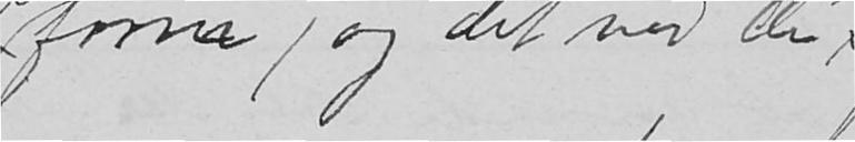form, og det ved de
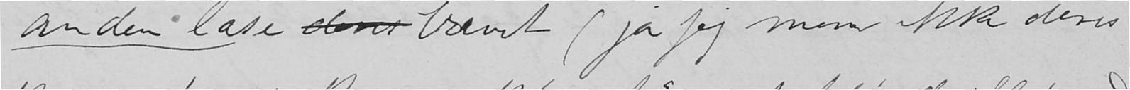anden læse deres brevet (ja jeg mener ikke deres
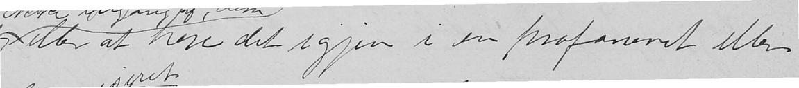eller at høre det igjen i en profaneret eller
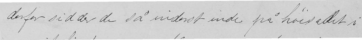derfor sidder de så inderst inde på høisædet i
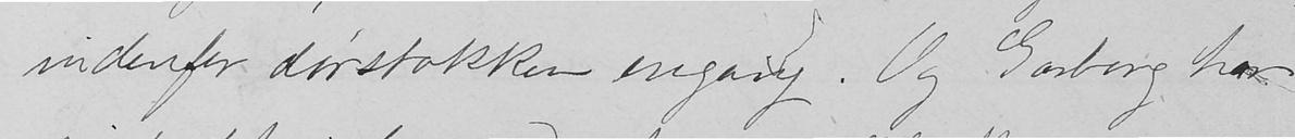indenfor dørstokken engang. Og Garborg har
